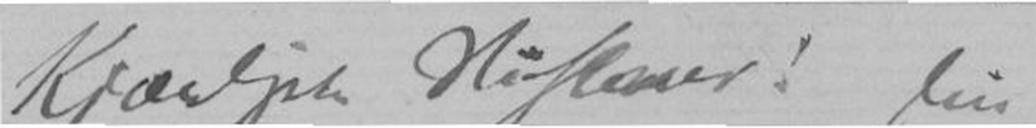Kjærligste Hilsener! Din
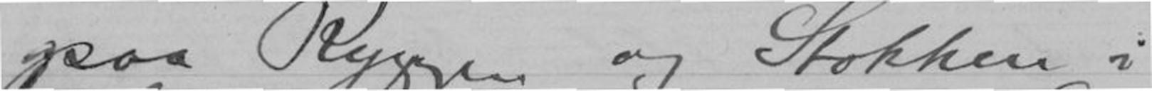paa Ryggen og Stokken i
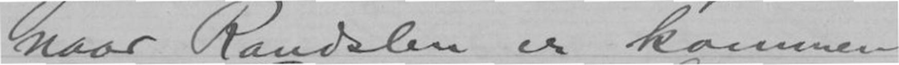naar Randslen er kommen
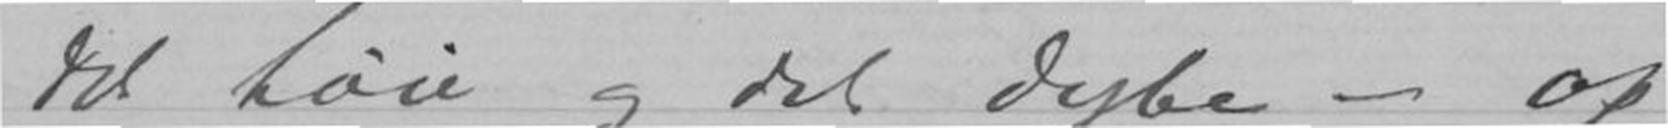det høie og det dybe - op
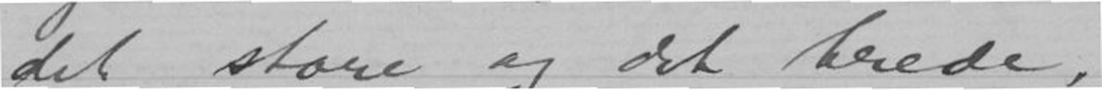det store og det brede,
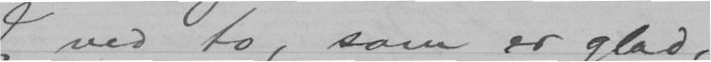Jeg ved to, som er glad,
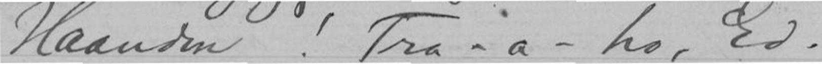Haanden! Tra-a-ho, Ed-
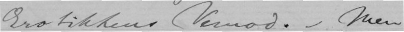Erotikkens Vemod. - Men
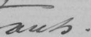Frants.
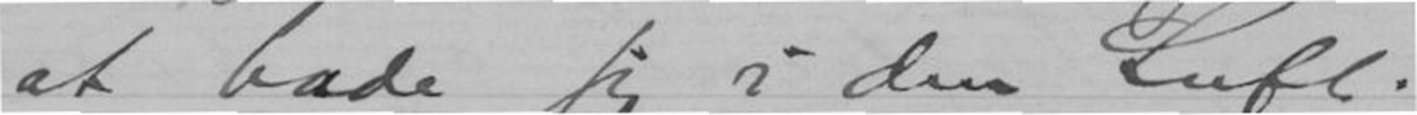at bade sig i den Luft.
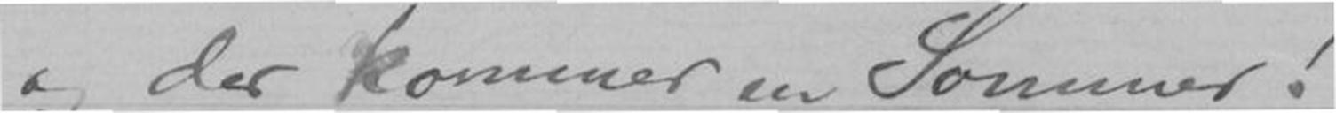og der kommer en Sommer!
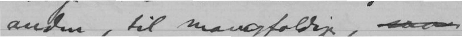anden, til mangfoldige, saa
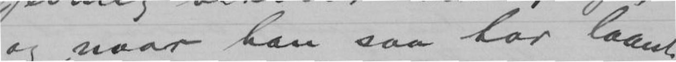og naar han saa har laant
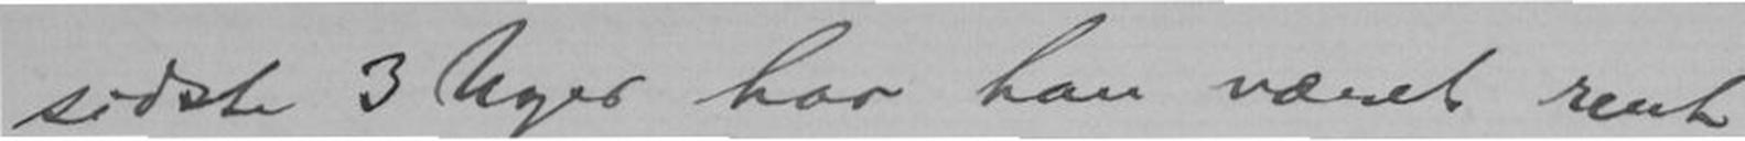sidste 3 Uger har han været rent
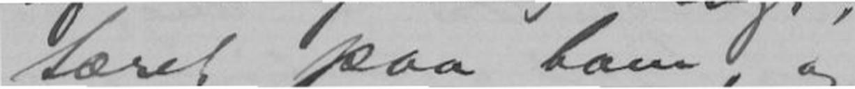tæret paa ham, og
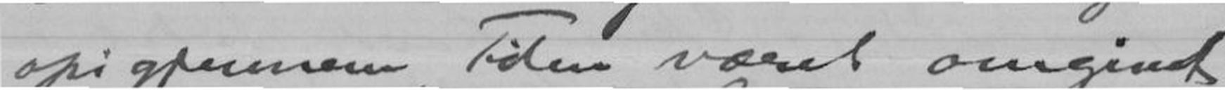opigjennem Tiden været omgivet
